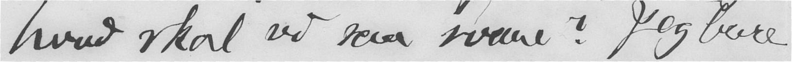hvad skal vi saa svare? Jeg bare
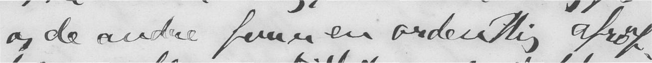og de andre faar en ordentlig afrøf-
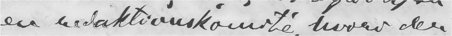en redaktionskomité, hvori der
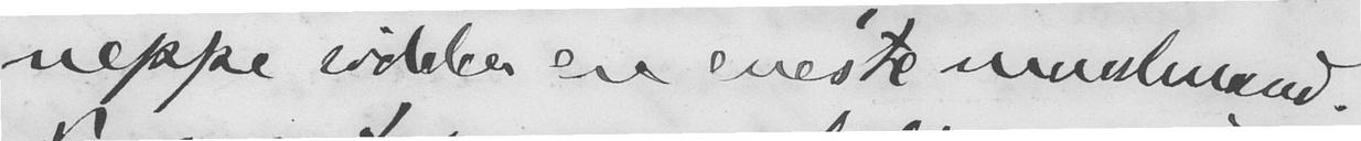neppe sidder en eneste maalmand.
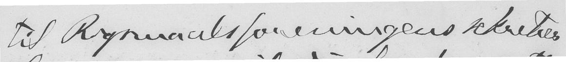til Rigsmaalsforeningens sekretær
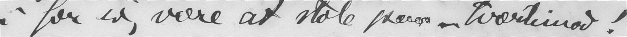i for sig være at stole paa - tværtimod!
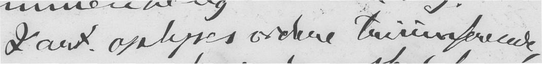I art. oplyses videre triumferende,
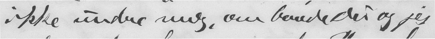ikke undre mig, om baade Du og jeg
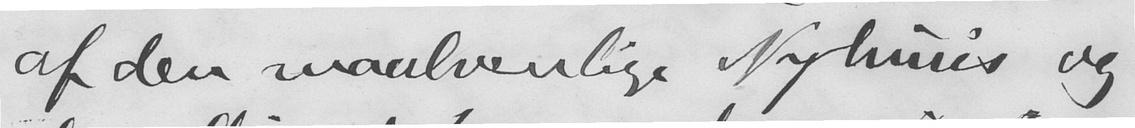af den maalvenlige Nyhuus og
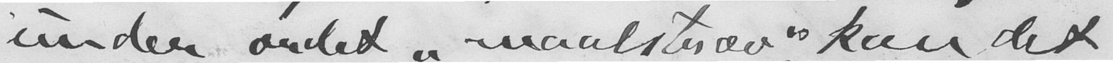under ordet "maalstræv", kan det
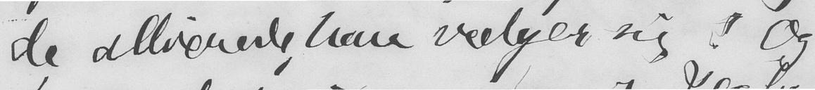de allierede, han vælger sig! Og
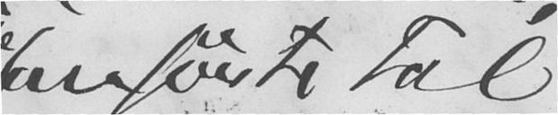anførte tal
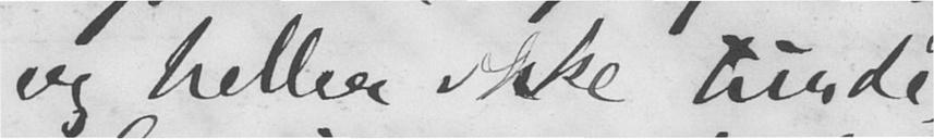og heller ikke turde
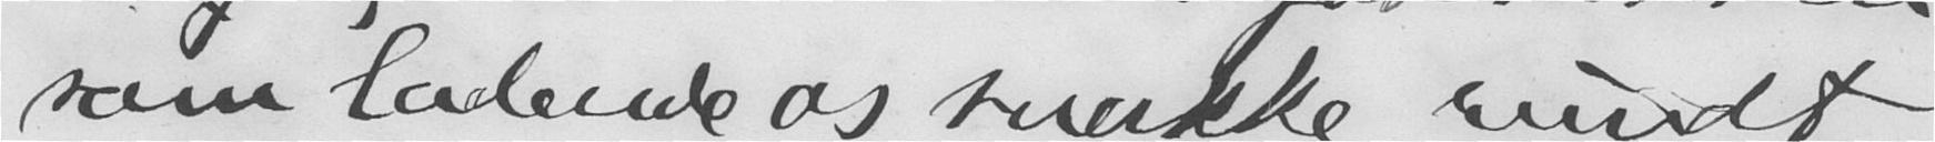som ladende os snakke rundt
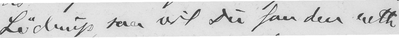Lødrup, saa vil Du faa den rette
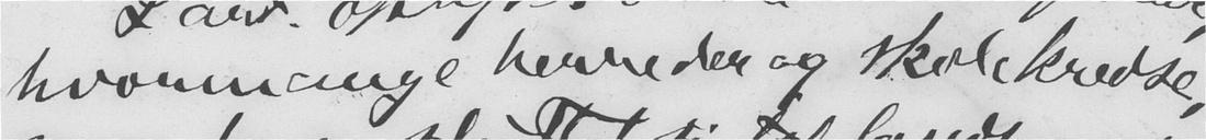hvormange herreder og skolekredse,
